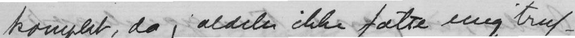komplet, da j aldeles ikke følte mig truf-
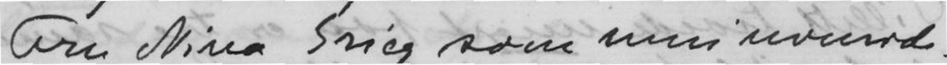Fru Nina Grieg som min uvurd-
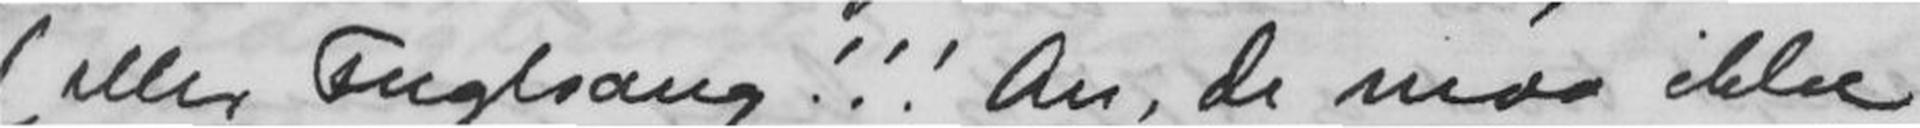(eller Fuglsang!!! Au, de maa ikke
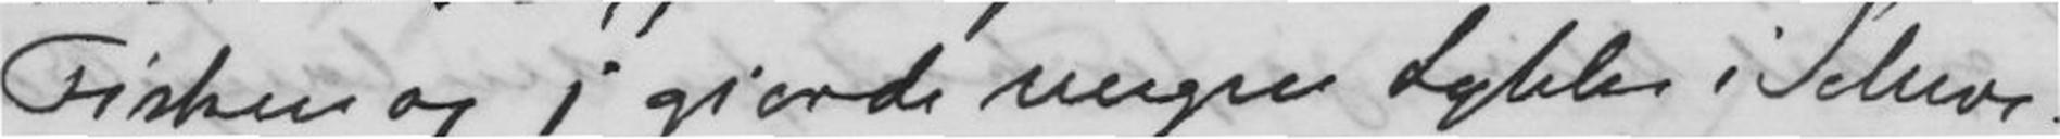Fisken og j giorde megen Lykke i Schwe-
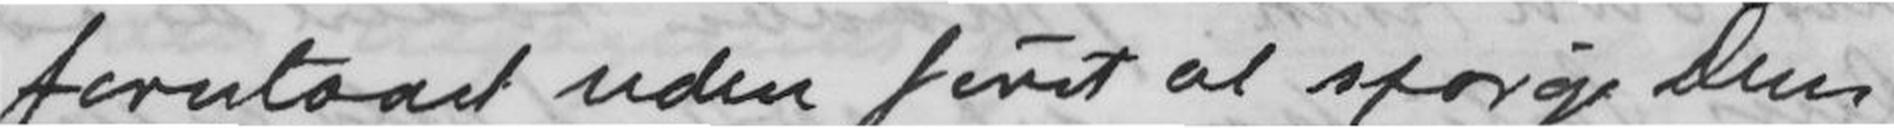forestaaet uden først at spørge Dem
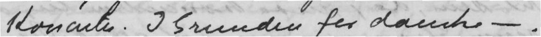Koncerte. I Grunden for danske -.
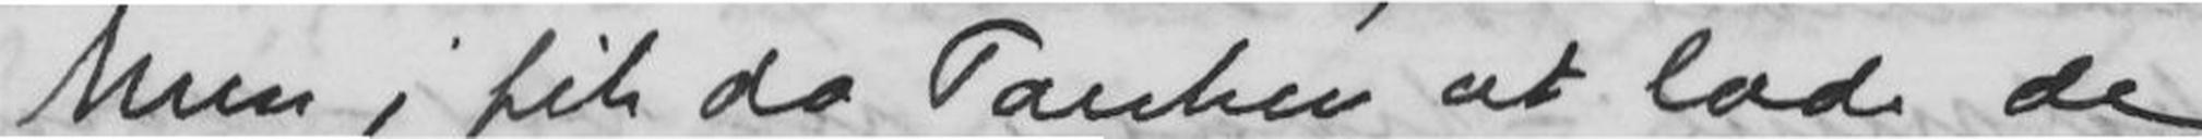Men j fik da Tanken at lade de
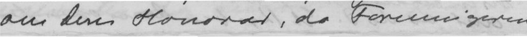om Deres Honorar, da Foreningeren
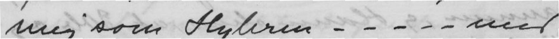mig som Hyleren ----- med
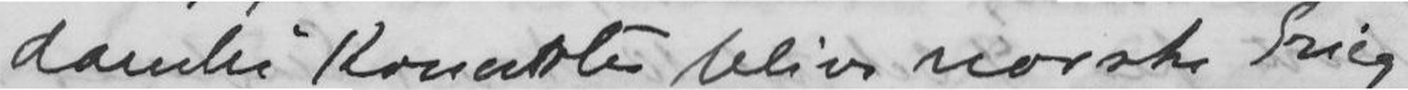danske Koncerte blive norske Grieg
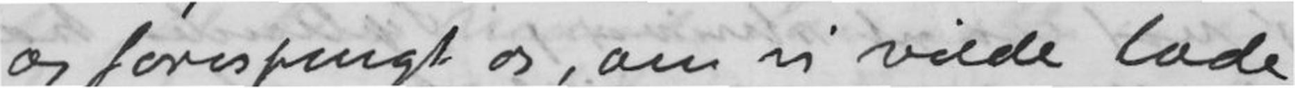og forespurgt os, om vi vilde lade
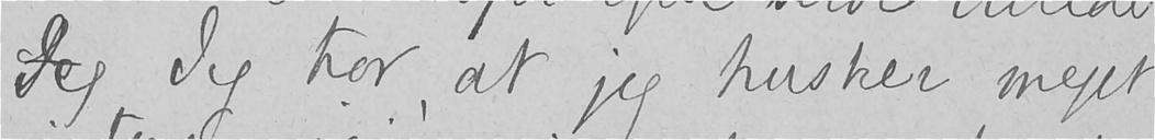Jeg Jeg tror, at jeg husker meget
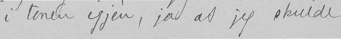i tonen igjen, ja at jeg skulde
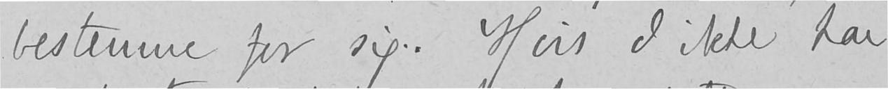bestemme for sig. Hvis I ikke har
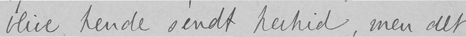blive hende sendt hertid, men det
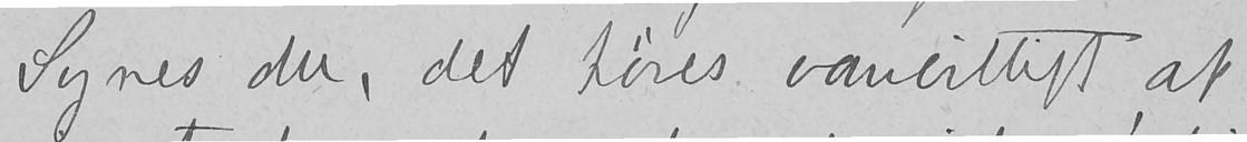Synes du, det høres vanvittigt at
